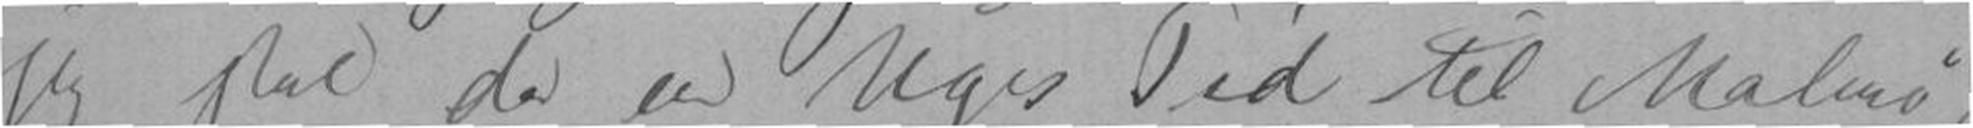jeg skal da en Uges Tid til Malmø,
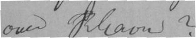over Khavn?
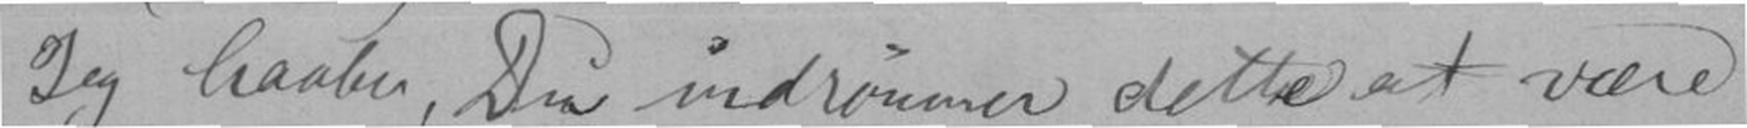Jeg haaber, Du indrømmer dette at være
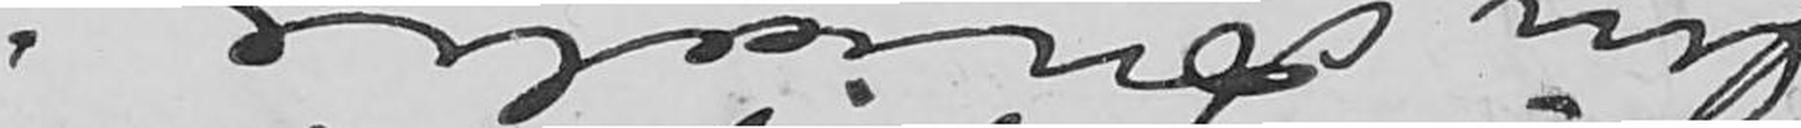Din Amalie.
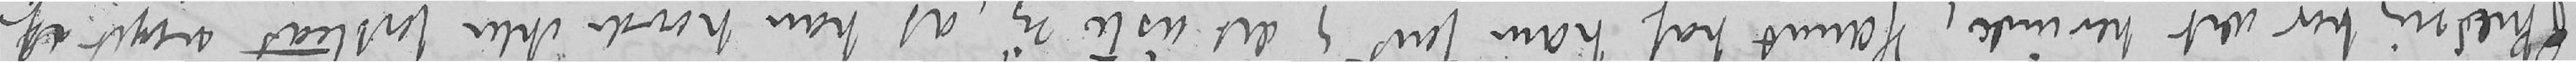Skredsvig har vært herinde, Harriet traf ham først og det viste sig, at han havde ikke forstaat noget af
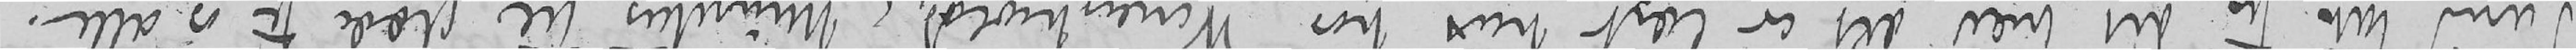Tusind tak for dit brev det er læst høit hos Werenskiolds, og Munthes til glæde for os alle.
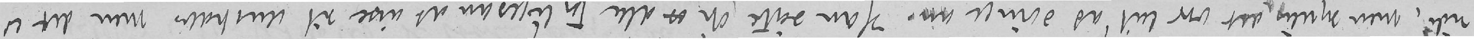side, men syntes det var leit at svinge nu. Han søgte op os alle for ligesom at vise sit venskab men det er
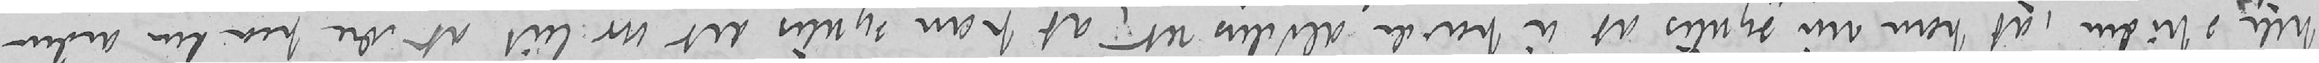hele striden, at han nu syntes at vi havde aldeles ret, at han syntes det var leit at være paa den anden
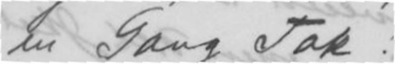en Gang Tak !
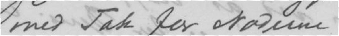med Tak for Noderne
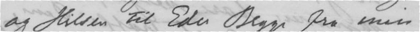og Hilsen til Eder Begge fra min
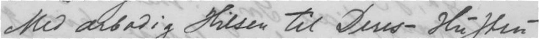Med ærbødig Hilsen til Deres- Hustru -
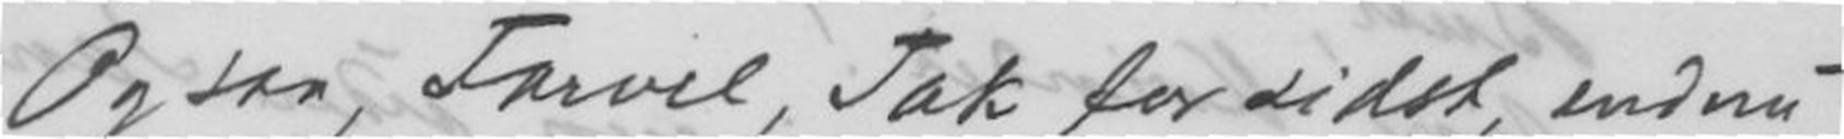Og saa, Farvel, Tak for sidst, endnu -
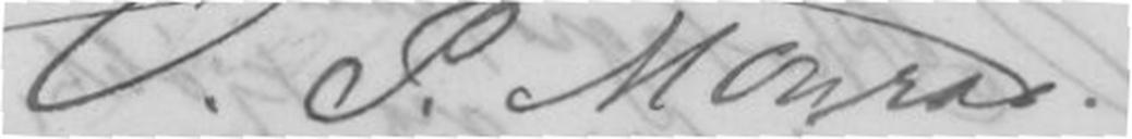O. P. Monrad.
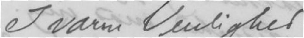I varm Venlighed
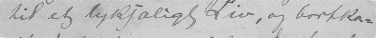til et lyksaligt Liv, og bortka-
